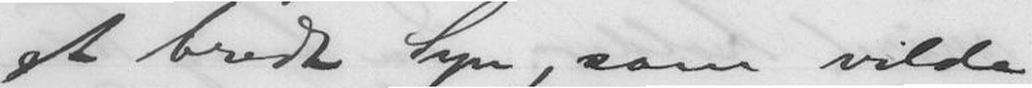et bredt Syn, som vilde
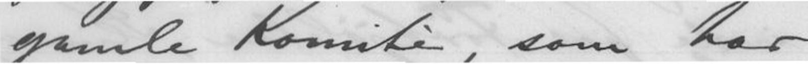gamle Komitè, som har
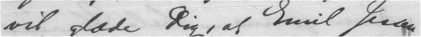vil glæde Dig, at Emil Jessen
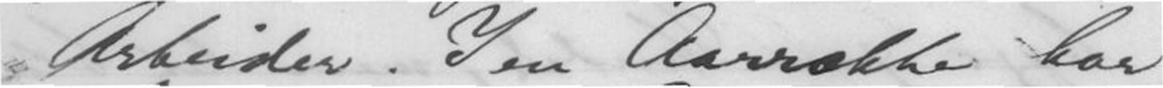Arbeider. I en Aarække har
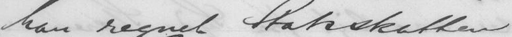han regnet Statsskatten
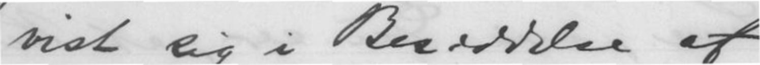vist sig i Besiddelse af
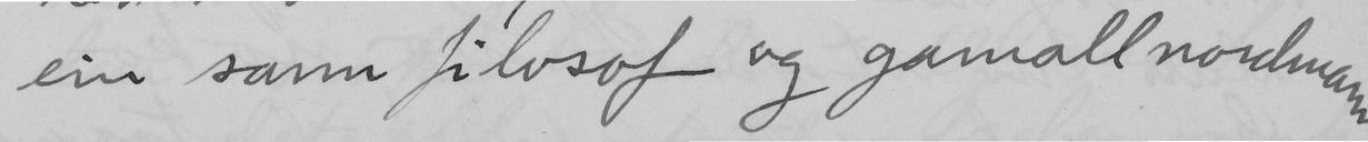ein sann filosof og gamall nordman.
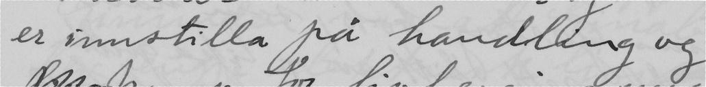er innstilla på handling og
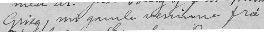Grieg, mi gamle venine frå
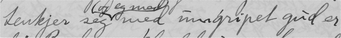tenkjer seg og eg med med umgripet gud er
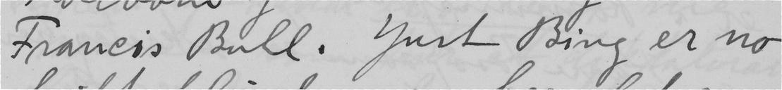Francis Bull. Just Bing er no
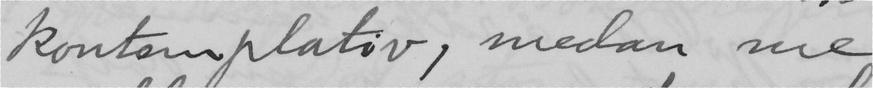kontemplativ, medan me
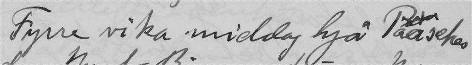Fyrre vika middag hjå Paasches
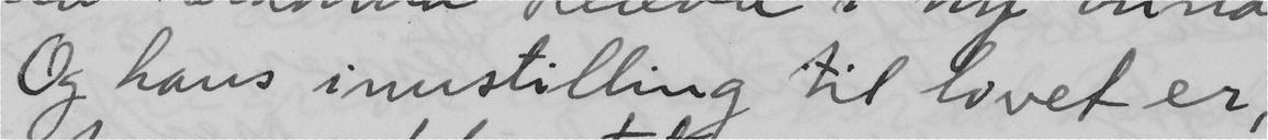Og hans innstilling til livet er,
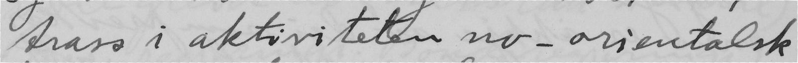trass i aktiviteten no – orientalsk
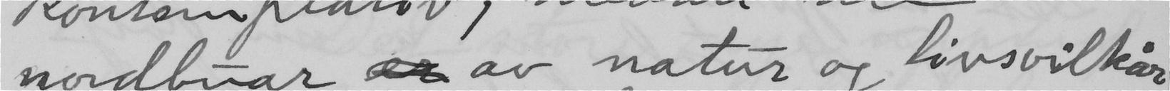nordbuar  av natur og livsvilkår
